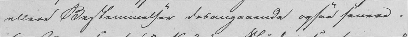ellere Bestemmelser desangaaende ogsaa senere.
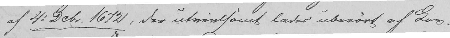af 4. Dcbr. 1672, der utvivlsomt lades uberørt af Lov-
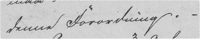denne Forordning. -
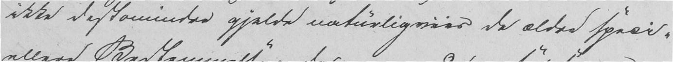ikke destomindre gjelde naturligviis de ældre speci-
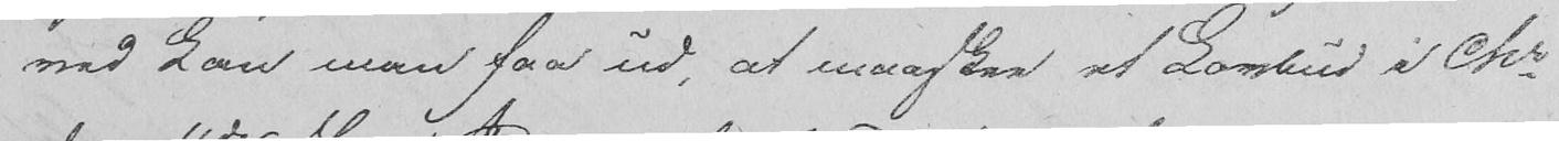ved kan man faa ud, at maaskee et Lovbud i Chr
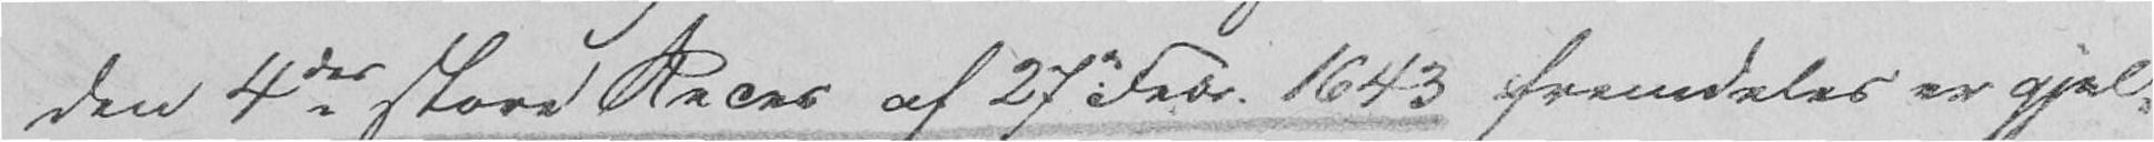den 4des store Reces af 27 Febr. 1643 fremdeles er gjel-
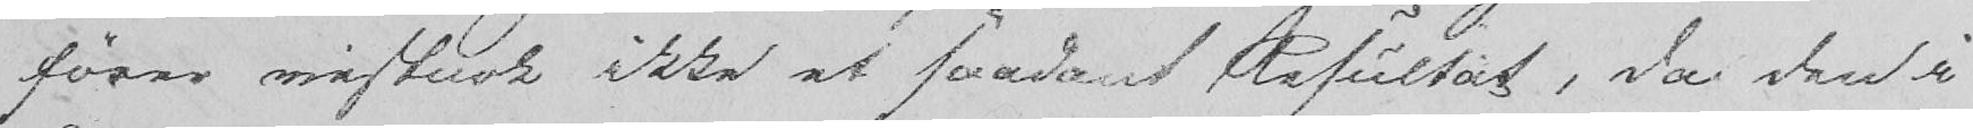fører vistnok ikke et saadant Resultat, da den i
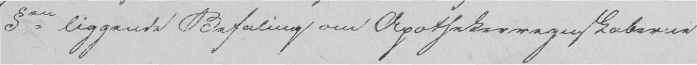§en liggende Befaling om Apothekerregnskaberne
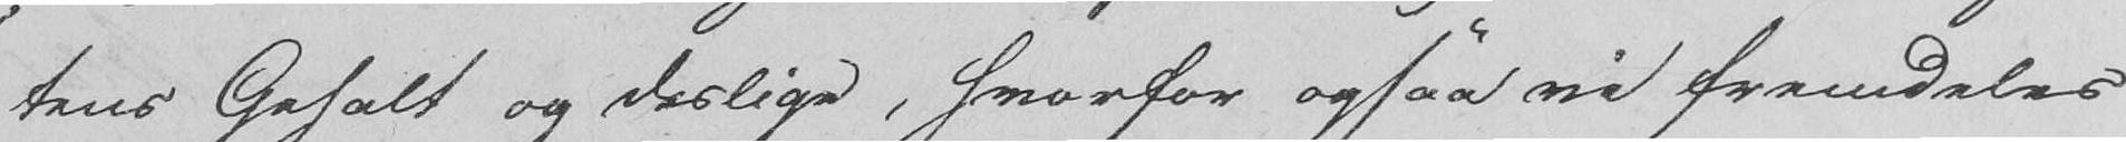tens Gehalt og deslige, hvorfor ogsaa vi fremdeles
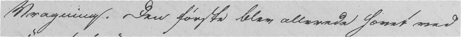Vragning. Den første blev allerede hævet ved
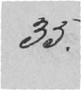35.
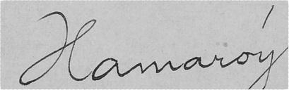Hamarøy
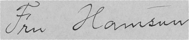Fru Hamsun
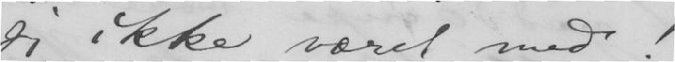jeg ikke været med!
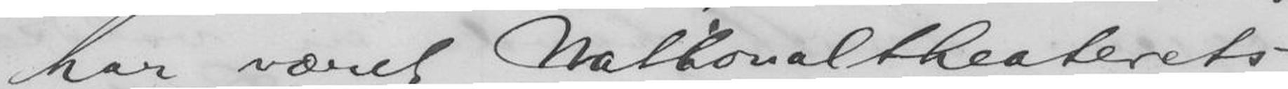har været Nationaltheaterets
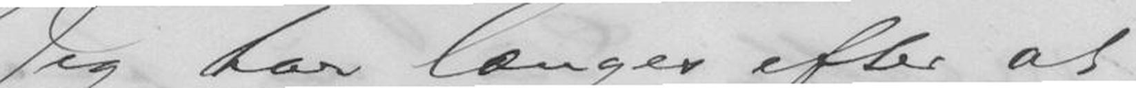Jeg har længes efter at
